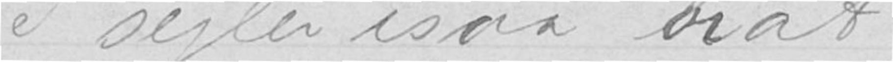"I sejler isaa brat,
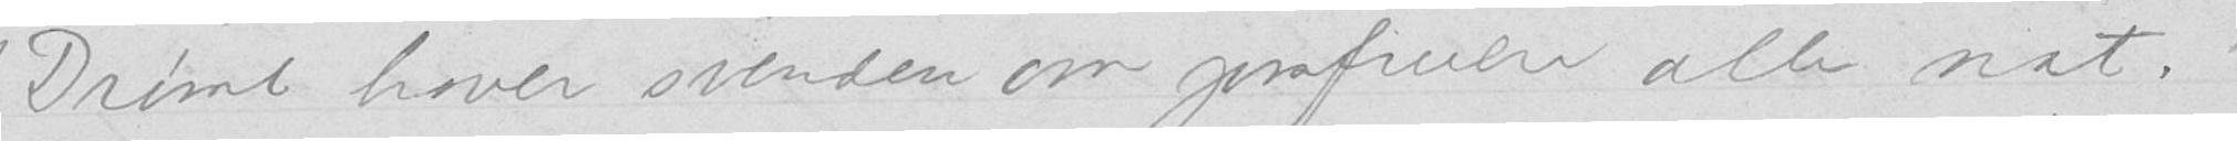"Drømt haver svenden om jomfruen alle nat."
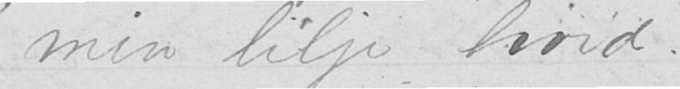"min lilje hvid.
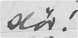slør.
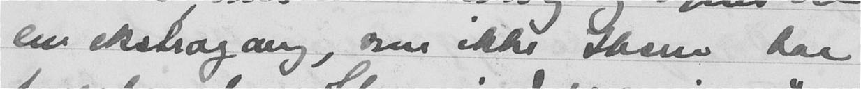en ekstragang, som ikke Ibsen har
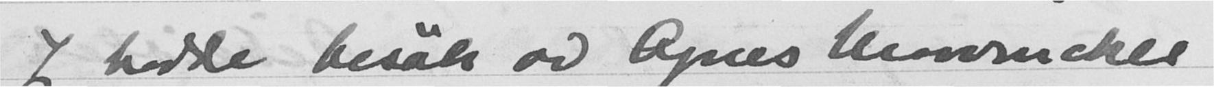Jeg hadde besøk av Agnes Mowinckel
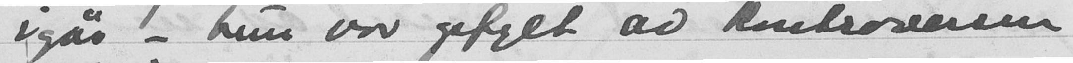igår - hun var opfylt av kontroversen
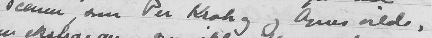scenen som Per Krohg og Agnes vilde,
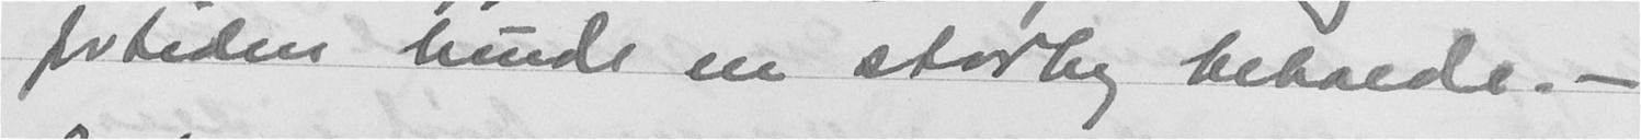fortiden burde en storby beholde. -
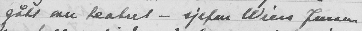gått over teatret - sjefen Wiers Jensen
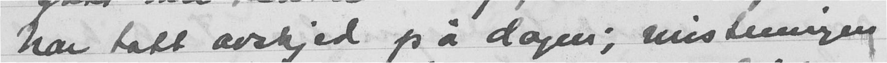har tatt avskjed p å dagen; misstemingen
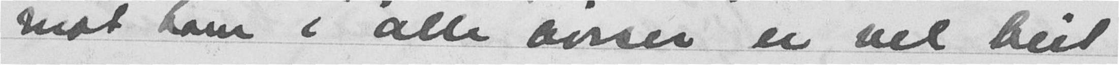mot ham i alle aviser er vel blit
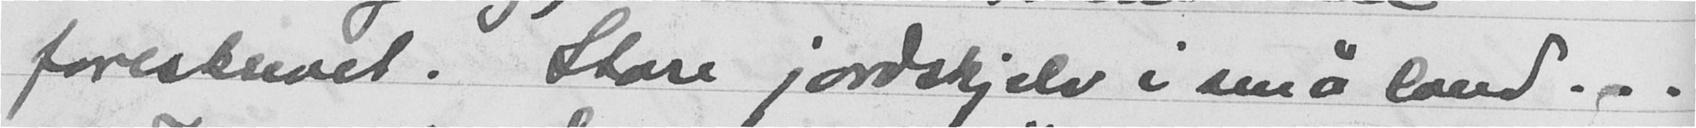foreskrevet. Store jordskjelv i små land...
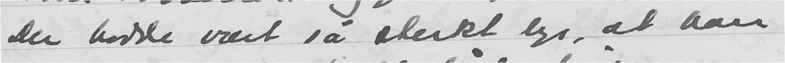Der hadde vært så sterkt lys, at han
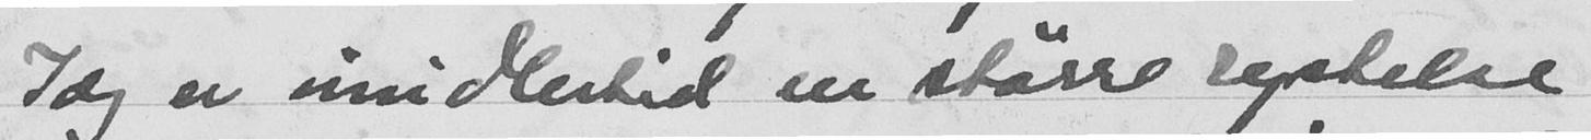Idag er imidlertid en større spøkelse
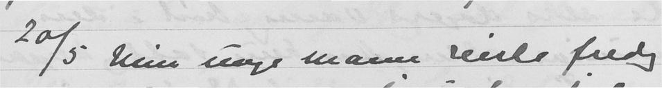20/5 Min unge mann reiste fredag
